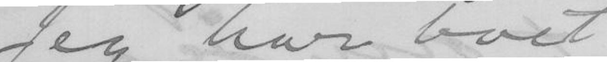Jeg har boet i
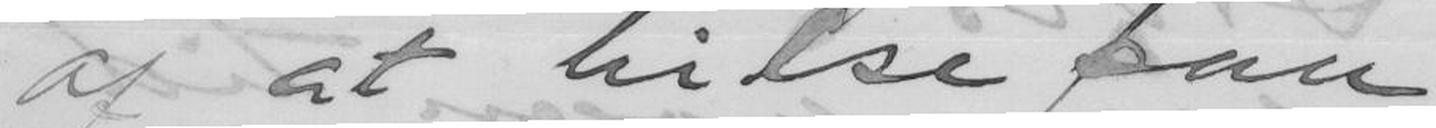af at hilse paa
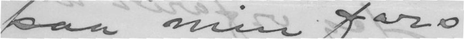paa min fars
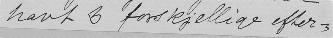havt 3 forskjellige efter-
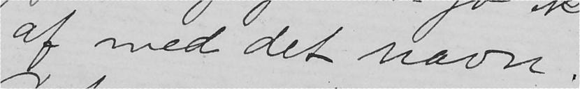af med det navn.
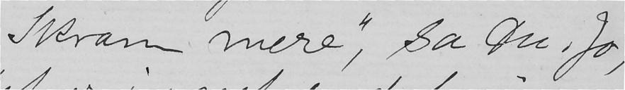Skram mere", sa Du. Jo,
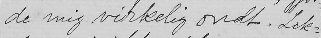de mig virkelig ondt. Lik-
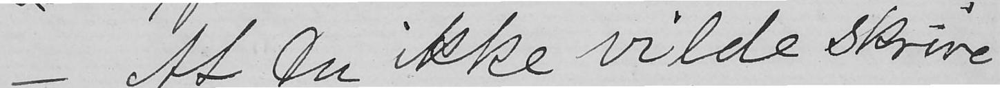- At Du ikke vilde skrive
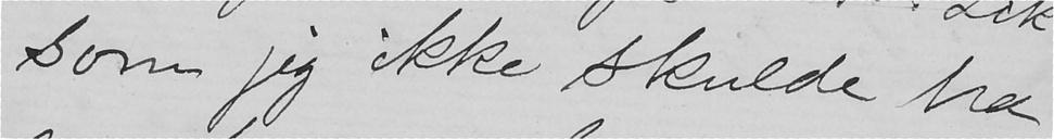som jeg ikke skulde ha
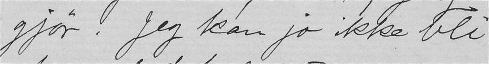gjør. Jeg kan jo ikke bli
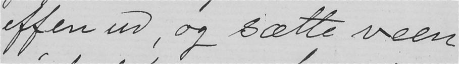effen ud, og sætte veen
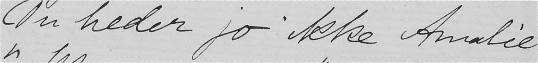"Du heder jo ikke Amalie
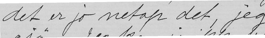det er jo netop det, jeg
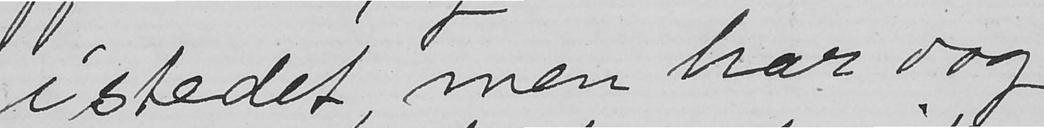i stedet, men har dog
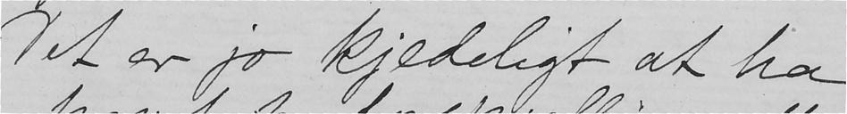Det er jo kjedeligt at ha
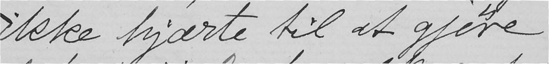ikke hjærte til at gjøre
